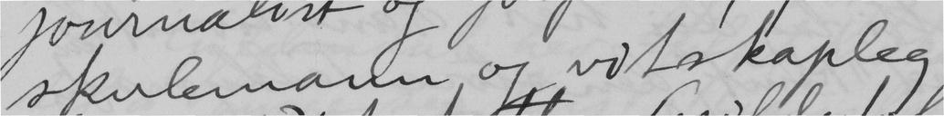skulemann og vitskapleg
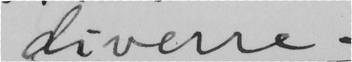diverre –
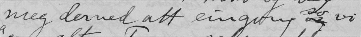meg der ned att eingong so vi
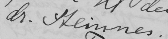dr. Steinnes.
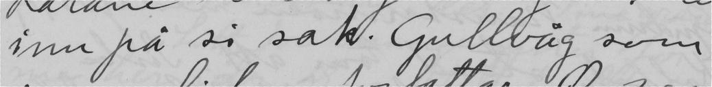inn på si sak. Gullvåg som
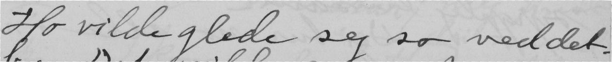Ho vilde glede seg so ved det.
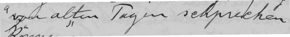"von alten Tagen schprechen
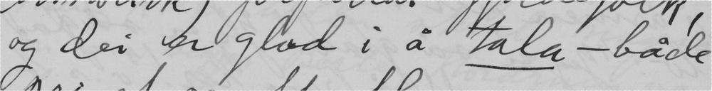og dei er glad i å tala – både
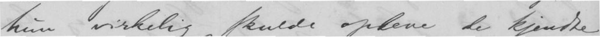hun virkelig skulde opleve de kjendte
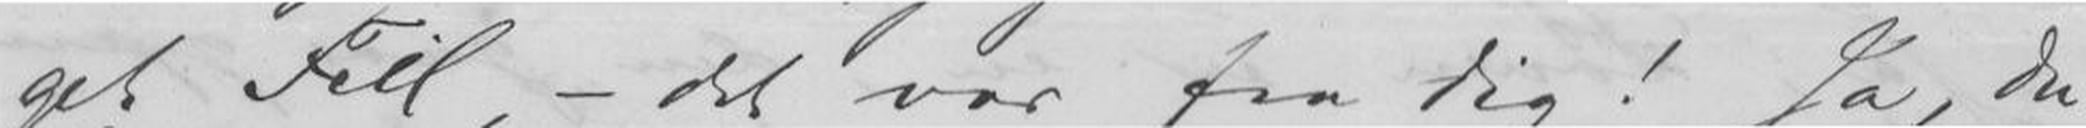get Feil, - det var fra Dig! Ja, Du
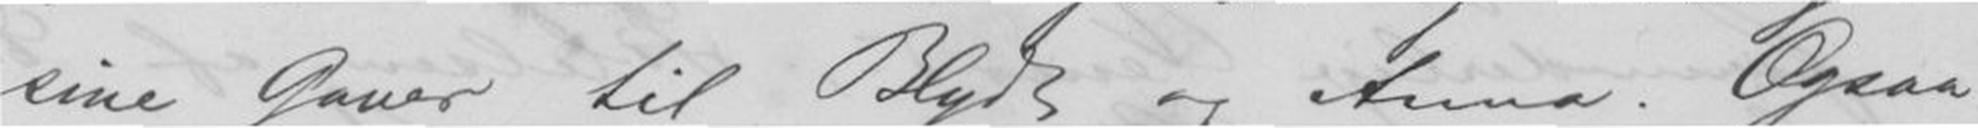sine Gaver til Blydt og Anna. Ogsaa
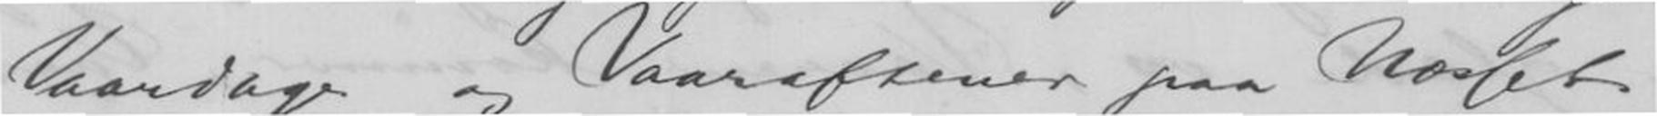Vaardage og Vaaraftener paa Næsset -
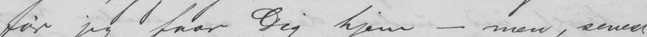før jeg faar Dig hjem - men, senest
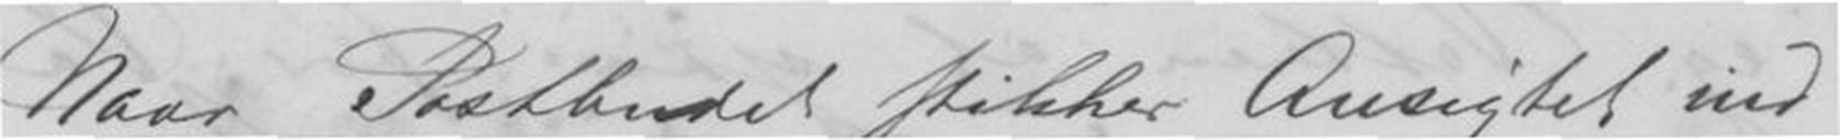Naar Postbudet stikker Ansigtet ind
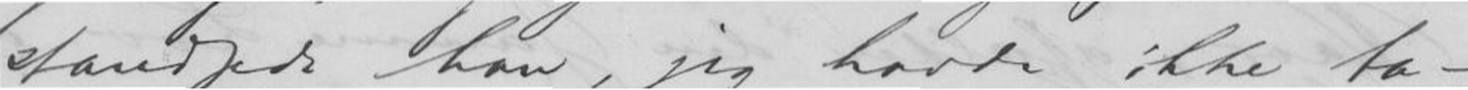standsede han, jeg havde ikke ta-
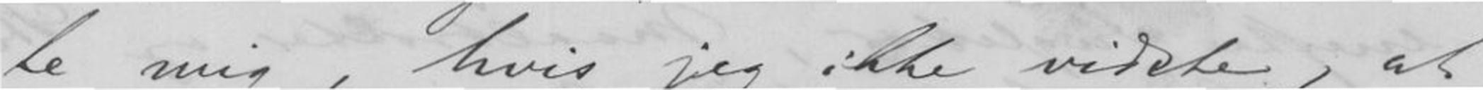te mig, hvis jeg ikke vidste, at
